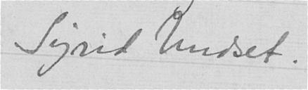Sigrid Undset.
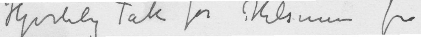Hjertelig Tak for Hilsenen fra
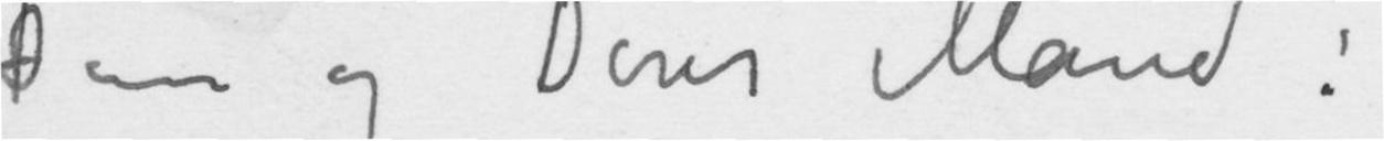Dem og Deres Mand!
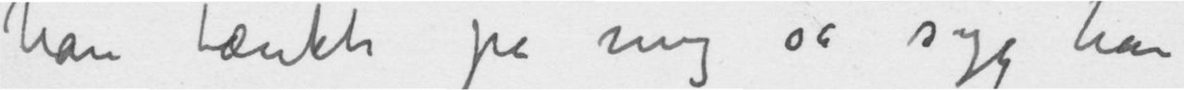han tænkte på mig så syg han
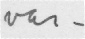var –
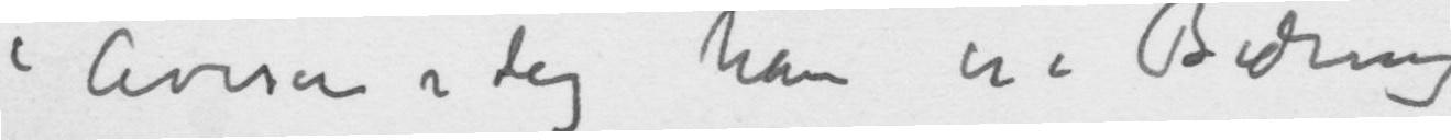i Avisen idag han er i Bedring
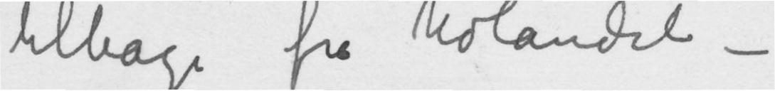tilbage fra Udlandet –
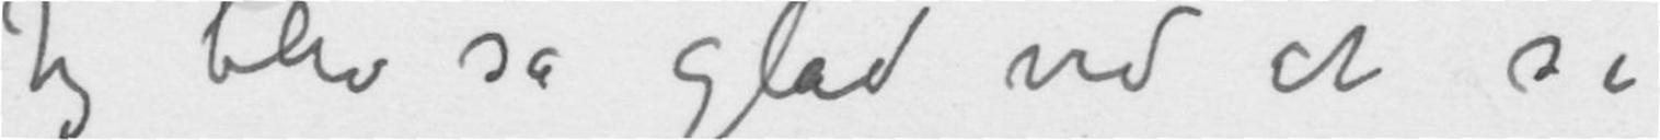Jeg blev så glad ved at se
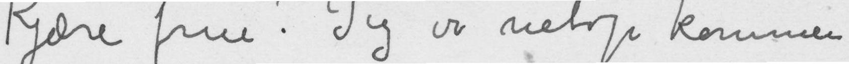Kjære frue! Jeg er netop kommen
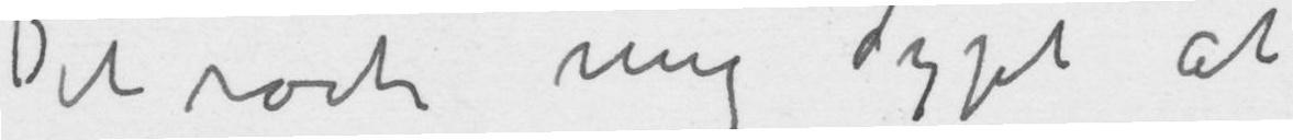Det rørte mig dypt at
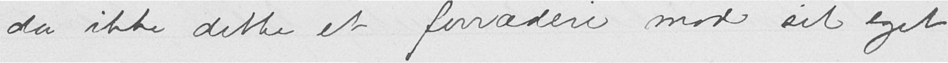da ikke dette et forræderi mod sit eget
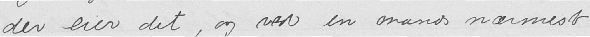der eier det, og ved en mands nærmest
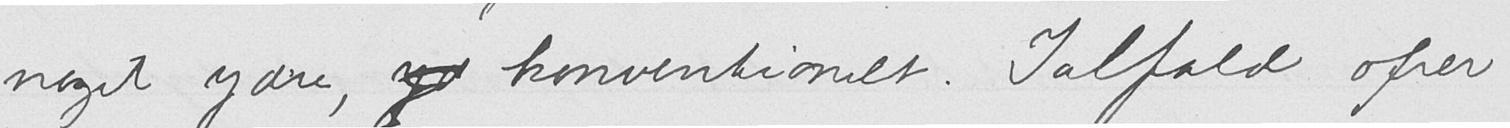noget ydre, og konventionelt. Ialfald ofrer
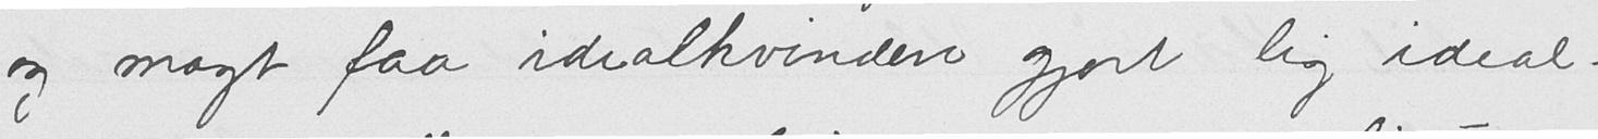og magt faa idealkvinden gjort lig ideal-
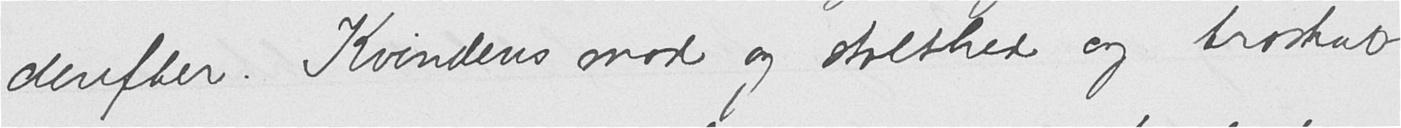derefter. Kvindens mod og stolthed og troskab
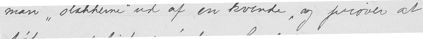man "slakkerne" ud af en kvinde, og prøver at
